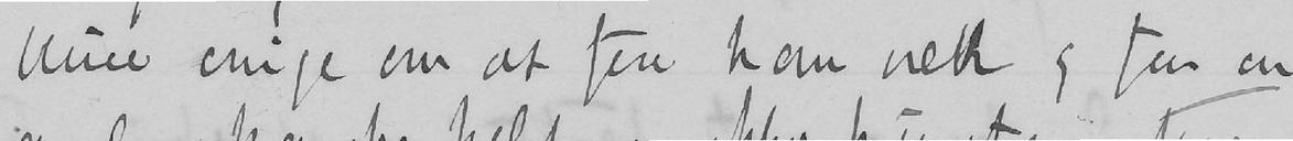blive enige om at faa ham væk og faa en
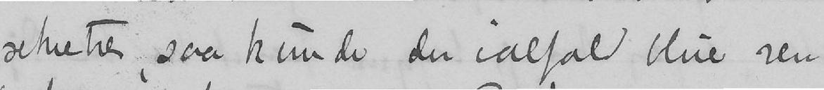sekretær, saa kunde der ialfald blive ren
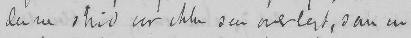denne strid var ikke saa overlagt, som en
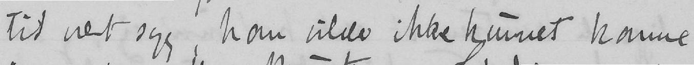tid vært syg, han vilde ikke kunnet komme
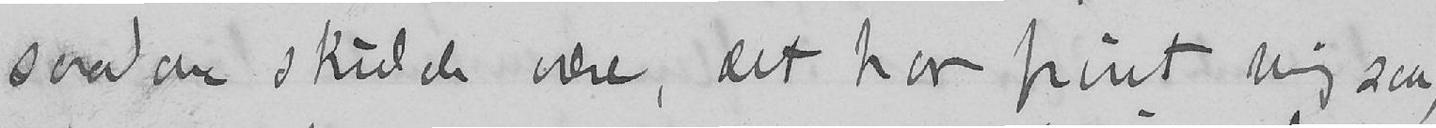saadan skulde være, det har pint mig saa,
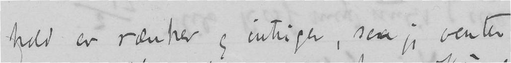hold er rænker og intriger, som jeg venter
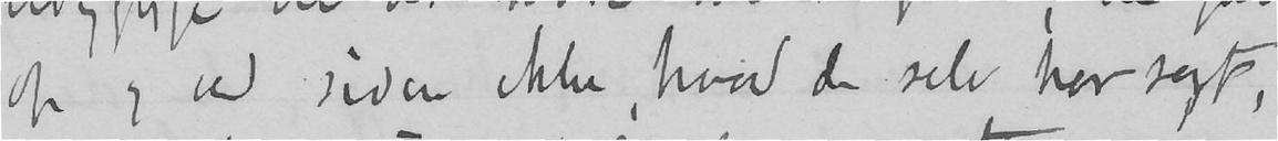op og ved siden ikke, hvad de selv har sagt,
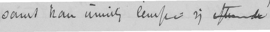samt kan umulig lempe sig efter de
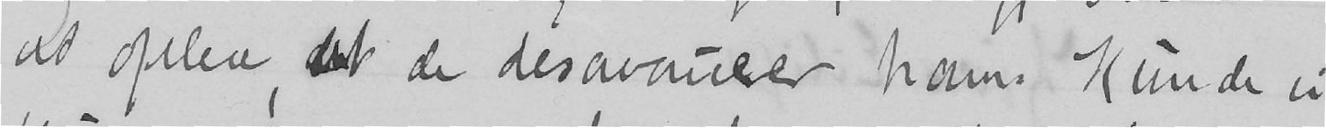at opleve, at de desavouerer ham. Kunde vi
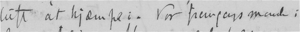luft at kjæmpe i. Vor fremgangsmaade i
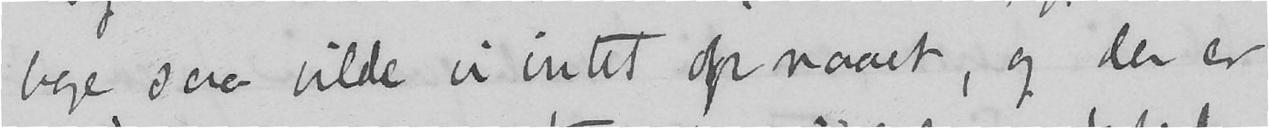bage, saa vilde vi intet opnaaet, og der er
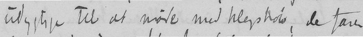udygtige til at møde med klagestik, de farer
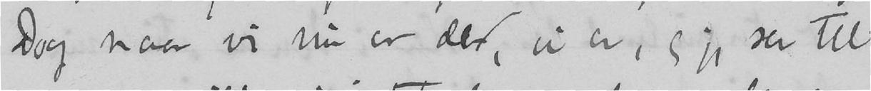Dog naar vi nu er der, vi er, og jeg ser til-
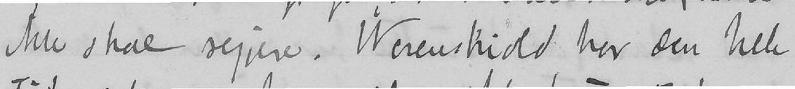ikke skal regjere. Werenskiold har den hele
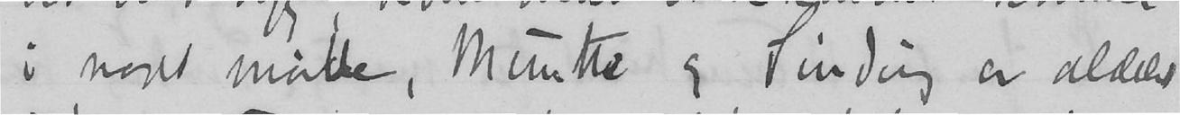i noget møde, Munthe og Sinding er aldeles
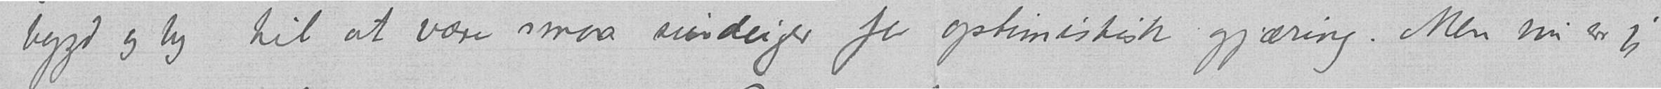bygd og by til at være smaa surdeiger for optimistisk gjæring. Men nu er jeg
